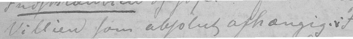Villien som absolut afhængig. I
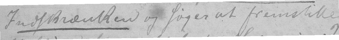Indskrænken og søger at fremstille
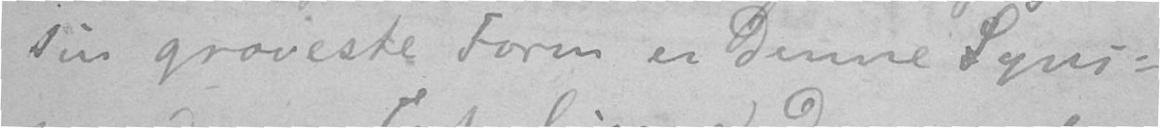sin groveste Form er denne Syns-
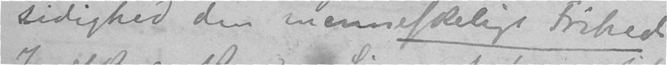sidighed den menneskelige Friheds
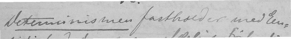Determinismen fastholder med Een-
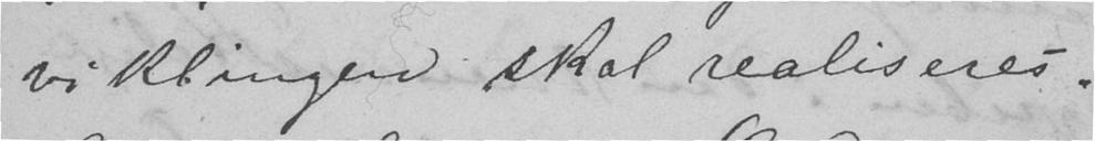viklingen skal realiseres.
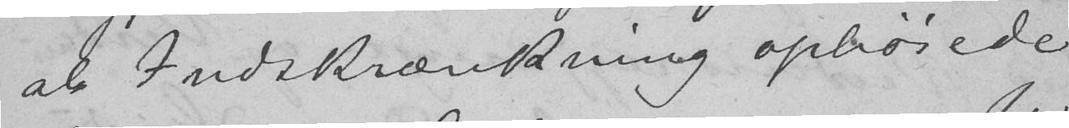al Indskrænkning ophøiede
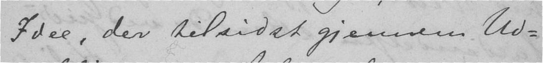Idee, der tilsidst gjennem Ud-
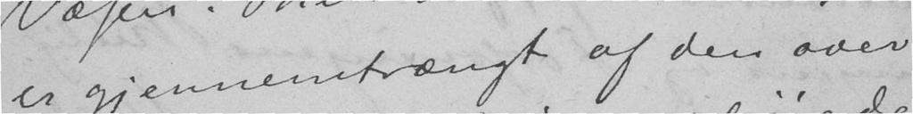er gjennemtrængt af den over
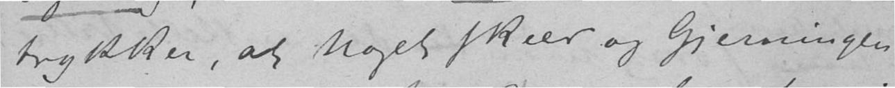trykker, at Noget skeer og Gjerningen
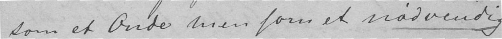som et Onde men som et nødvendig
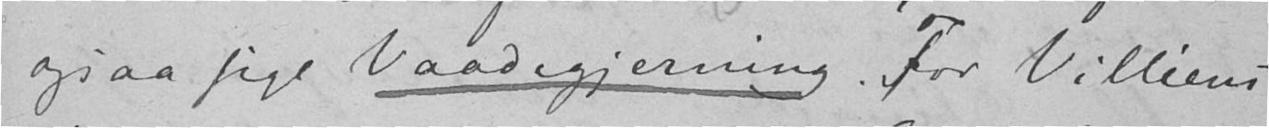ogsaa sige Vaadegjerning. For Villiens
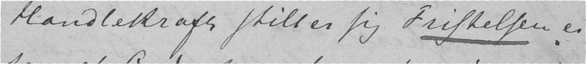Handlekraft stiller sig Fristelsen ei
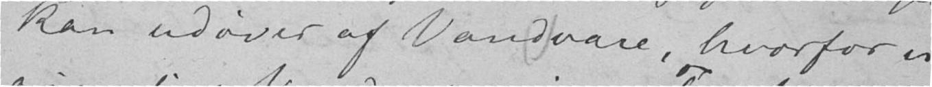kan udøves af Vandvare, hvorfor vi
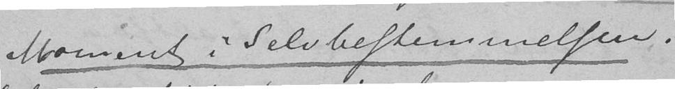Moment i Selvbestemmelsen.
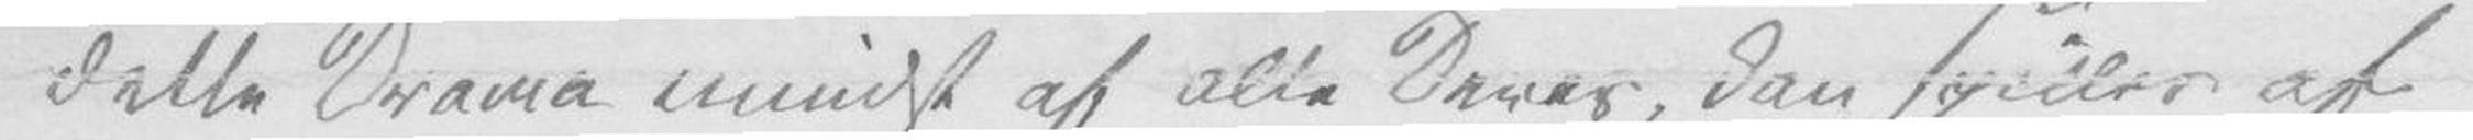dette Drama mindst af alle Deres, kan spilles af
